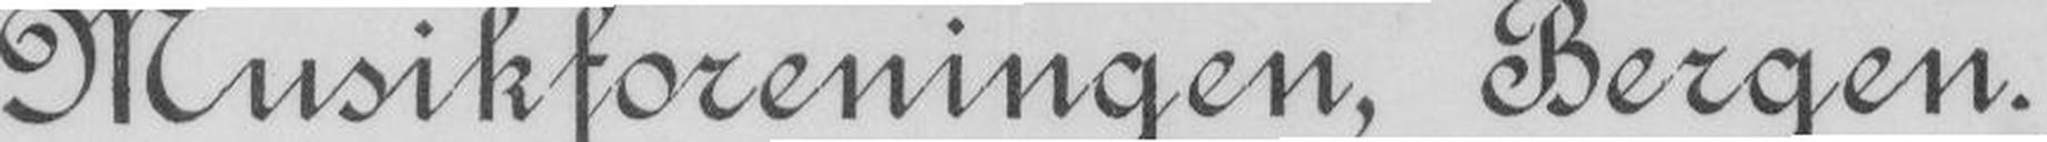Musikforeningen, Bergen.
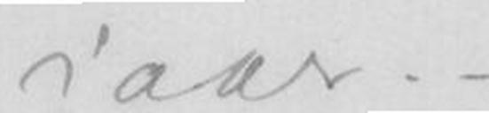iaar. -
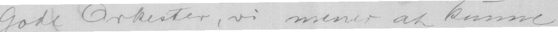Gode Orkester, vi mener at kunne
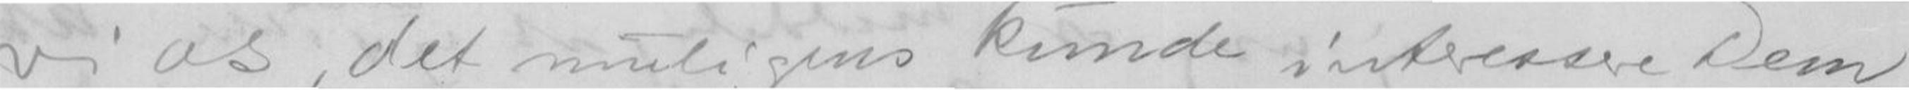vi os, det muligens kunde interessere Dem
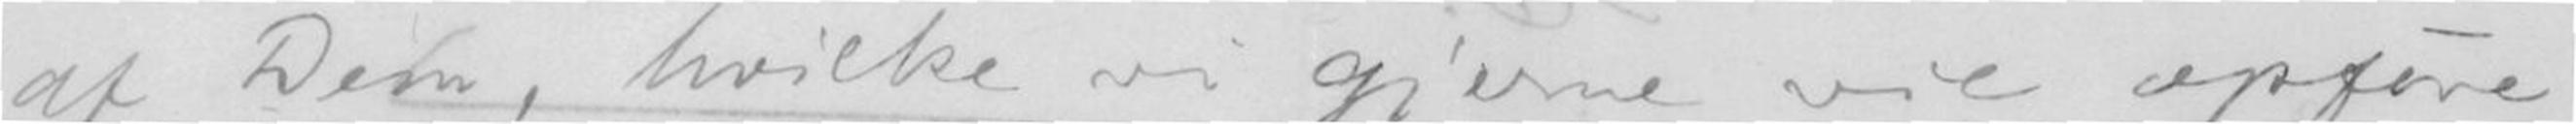af Dem, hvilke vi gjerne vil opføre
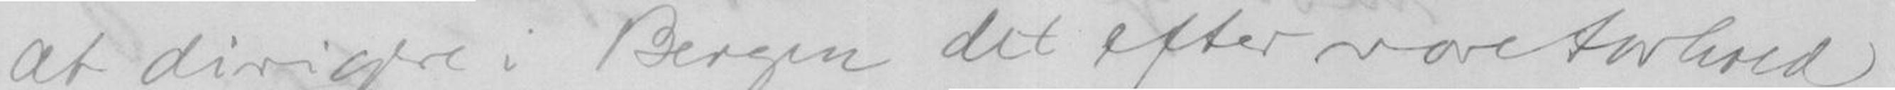at dirigere i Bergen det efter voreforhold
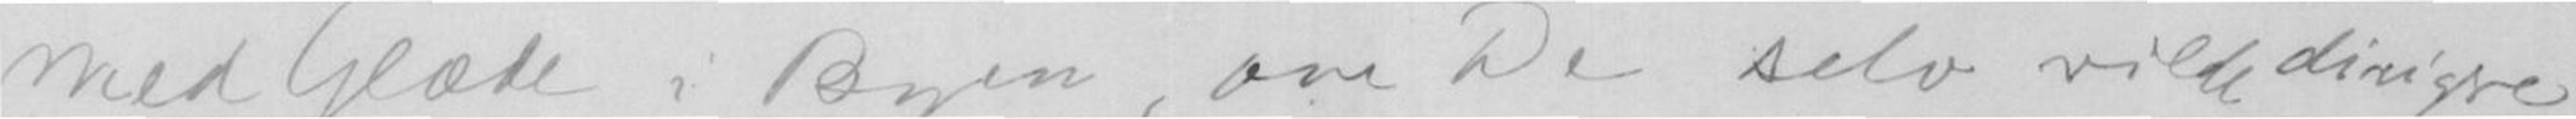med Glæde i Byen, om De selv vilde dirigere
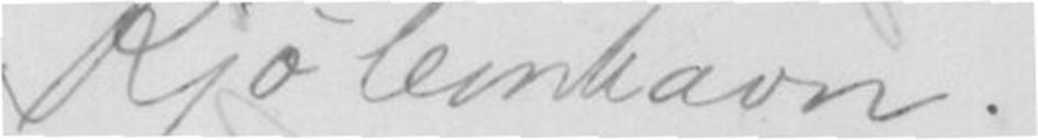Kjøbenhavn.
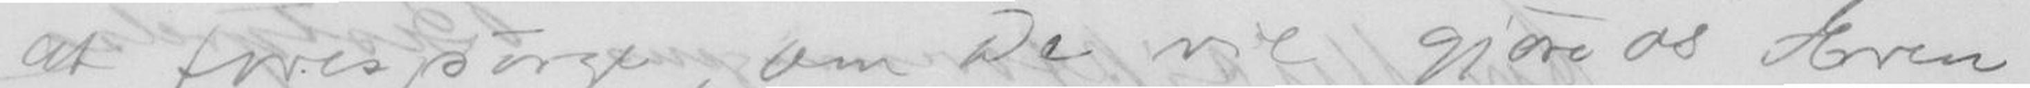at forespørge, om De vil gjøre os Æren
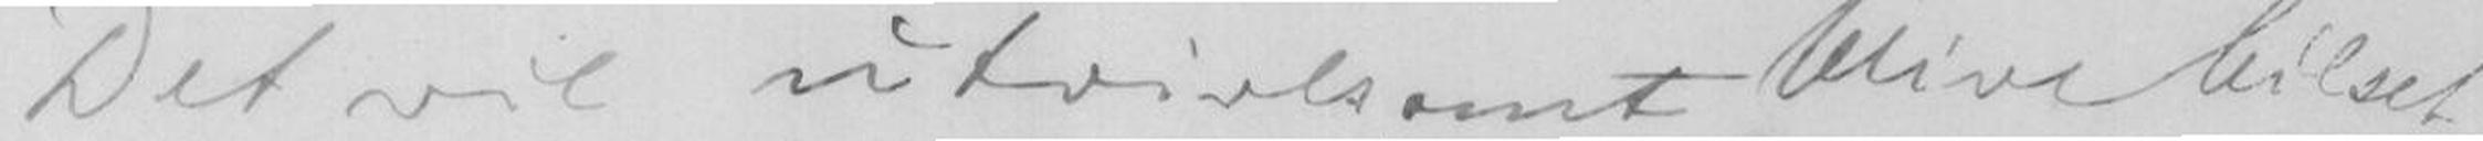Det vil utvivlsomt blive hilset
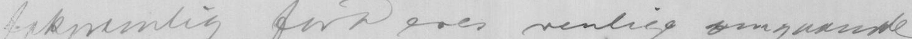taknemlig for Deres venlige omgaaende
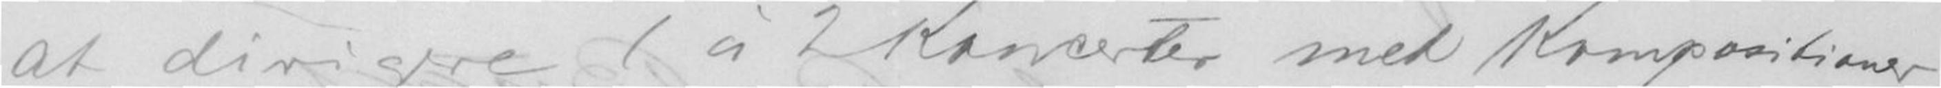at dirigere 1 à 2 Koncerter med Kompositioner
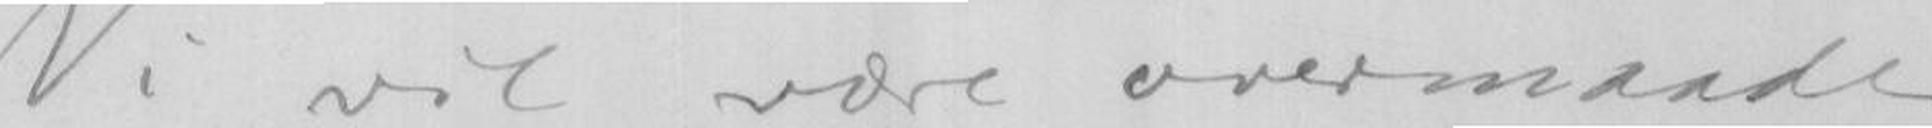Vi vil være overmaade
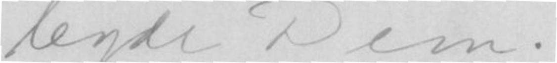byde Dem.
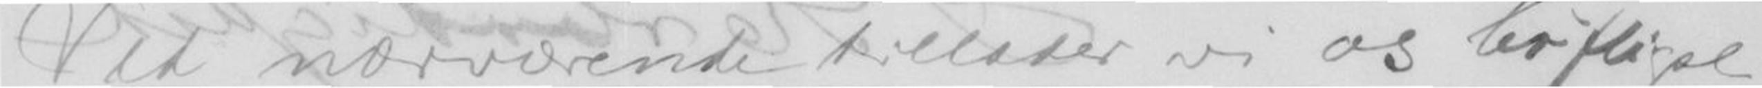Ved nærværende tillader vi os høfligst
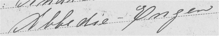Abbedie-Engen
